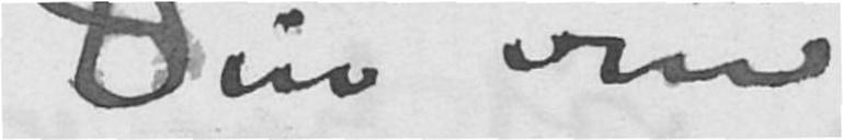Din ven
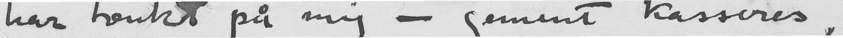har tænkt på mig - gement kasseres,
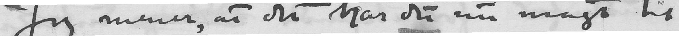Jeg mener, at det har du nu magt til
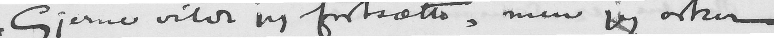Gjerne vilde jeg fortsætte, men jeg orker
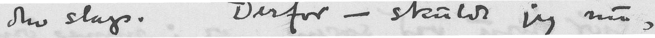den slags. Derfor - skulde jeg nu,
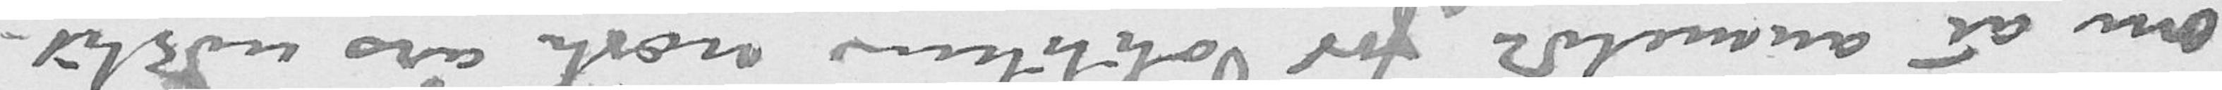om at anmelde for "Politiken" næste års udstil-
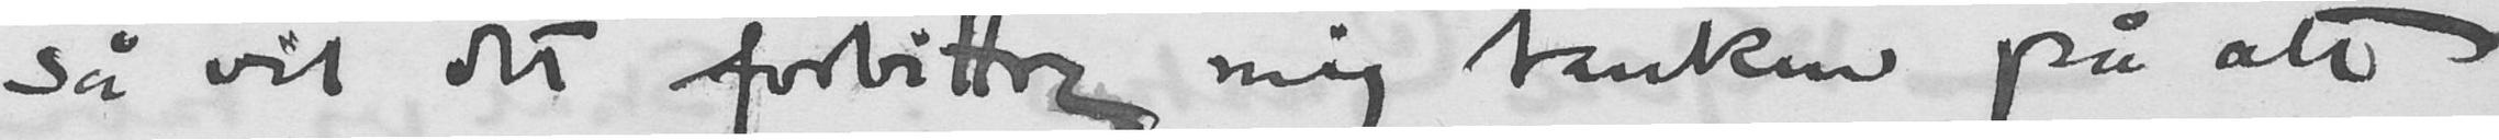så vil det forbittre mig tanken på alt
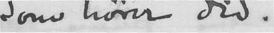som hører til.
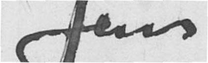Jens
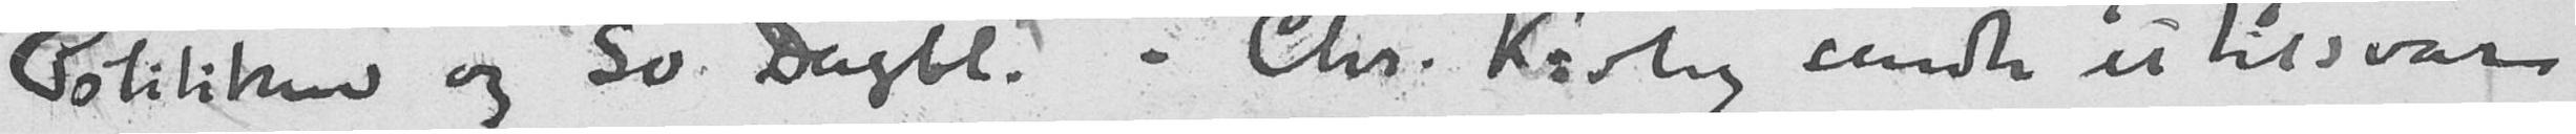"Politiken" og  "Sv. Dagbl." - Chr. Krohg sendte et tilsvar
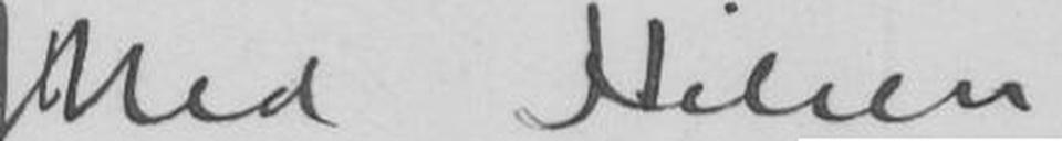Med Hilsen
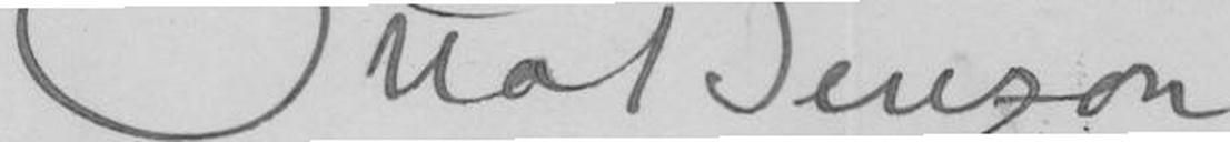Otto Benzon
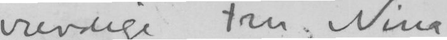værdige Fru Nina
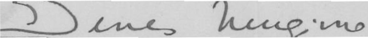Deres henginve
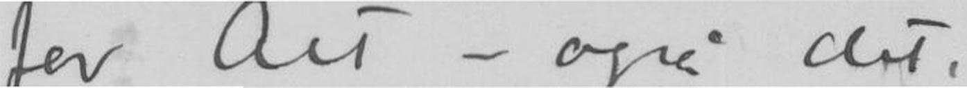for Alt - også det
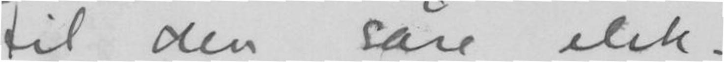til den såre elsk-
